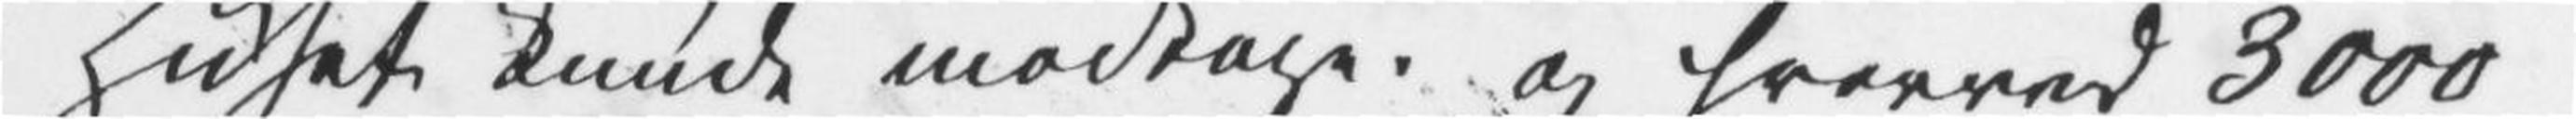Huset kunde modtage, og hvorved «3000
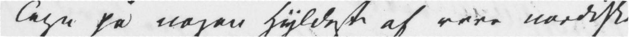Tegn på nogen Hyldest af vore «nordiske
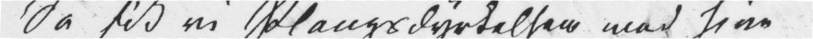Så fik vi Plougsdyrkelsen med sine
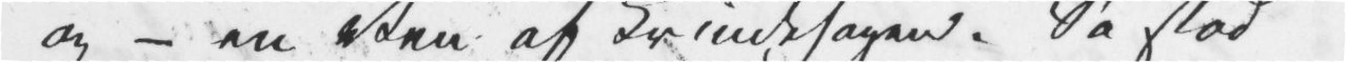og – en «Ven af Kvindesagen». Så stod
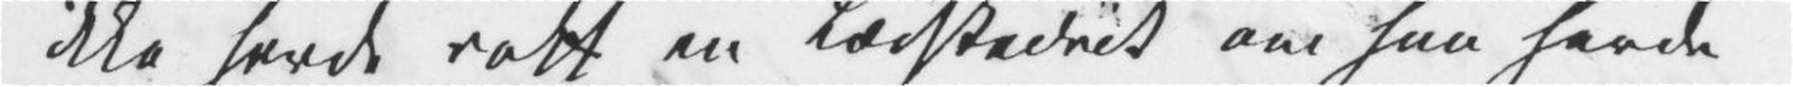ikke havde rakt en Lædskedrik om han havde
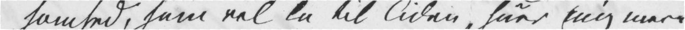somhed, som vel lå til Tiden, huer mig mere
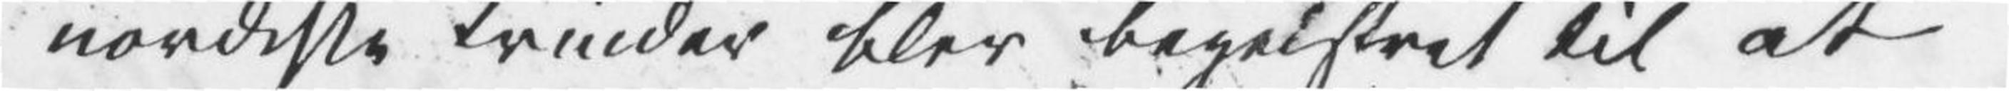nordiske Kvinder» blev begeistret til at
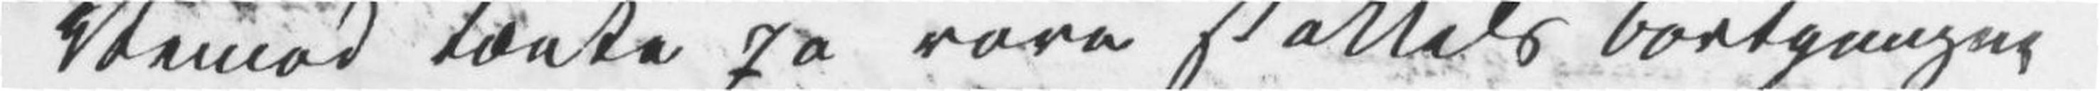Vemod tænke på vore stakkels bortgangne
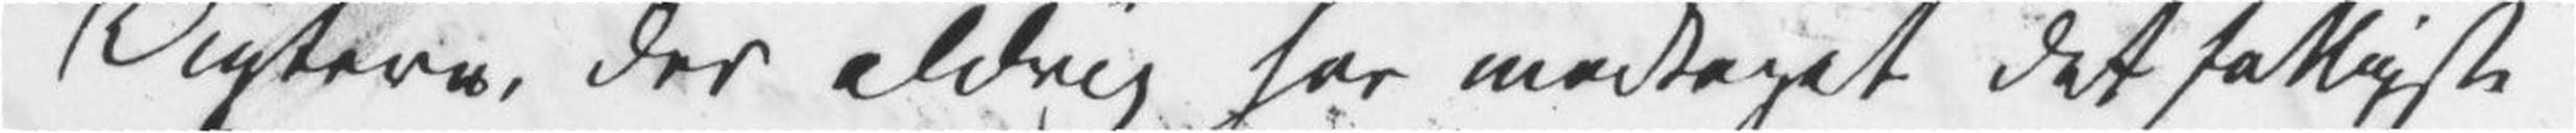Digtere, der aldrig har modtaget det fattigste
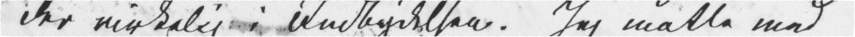der virkelig i Indbydelsen. Jeg måtte med
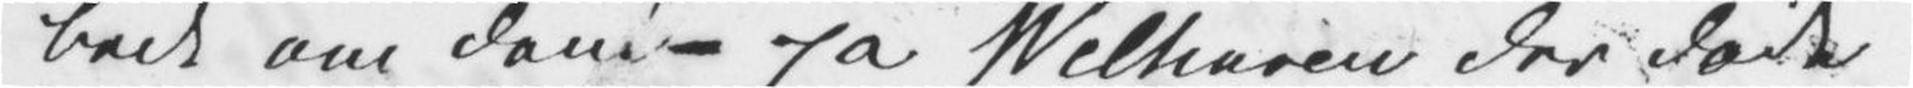bedt om den – på Welhaven der døde
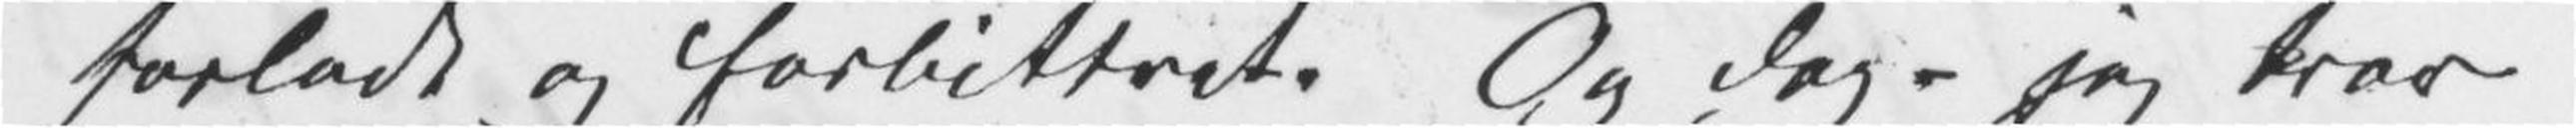forladt og forbittret. Og dog – jeg tror
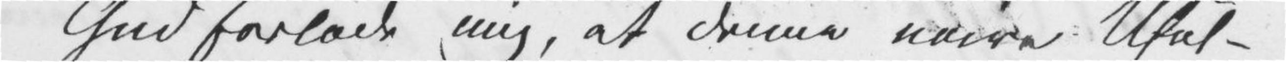Gud forlade mig, at denne naive Uføl¬
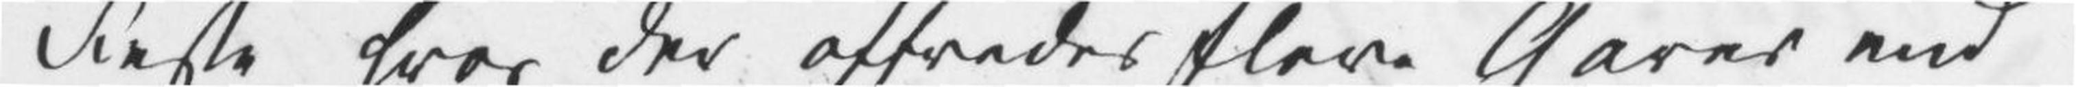Feste hvor der offredes flere Gaver end
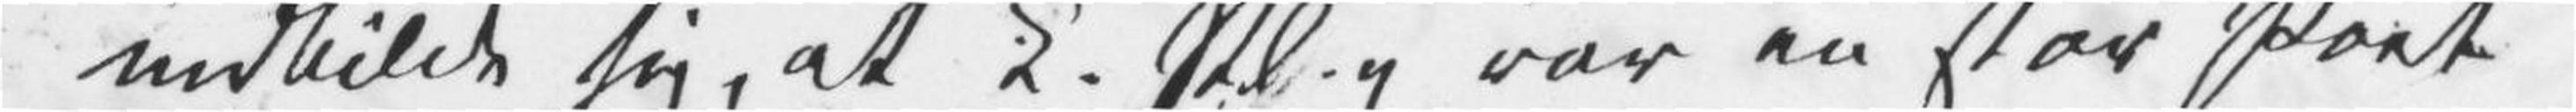indbilde sig, at C. Pl.g var en stor Poet
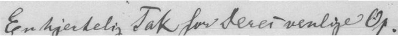En hjertelig Tak for Deres venlige Op-
